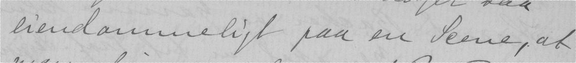eiendommeligt paa en Scene, at
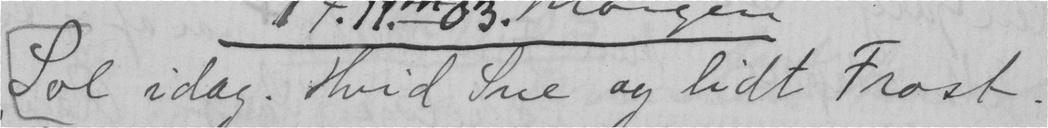Sol idag. Hvid sne og lidt Frost.
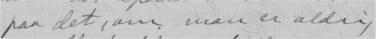paa det, om man er aldrig
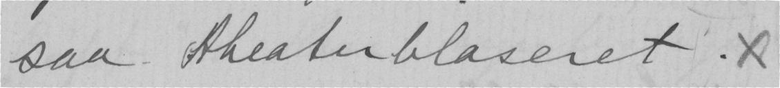saa theaterblaseret. x
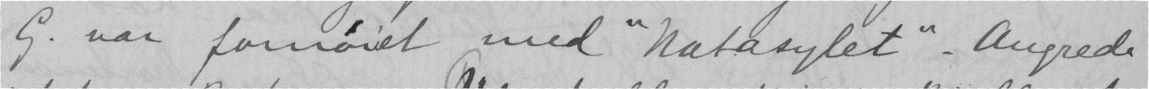G. var fornøiet med "Natasylet". Angrede
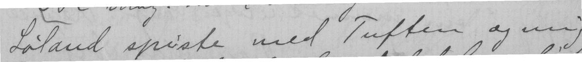Løland spiste med Tuften og mig
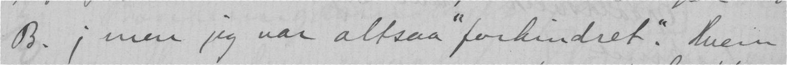B. ; men jeg var altsaa "forhindret". Hvem
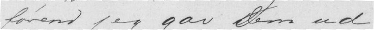førend jeg gav dem ud,
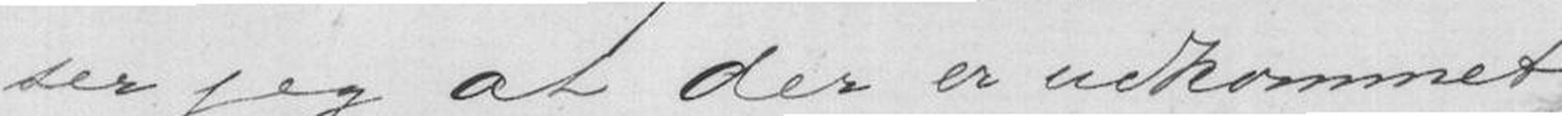ser jeg at der er udkommet
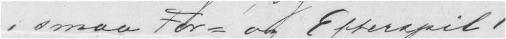i smaa For = og Efterspil
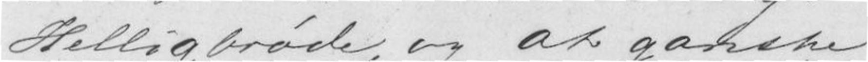Helligbrøde, og at ganske
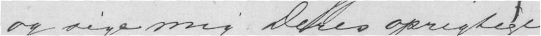og sige mig Deres oprigtige
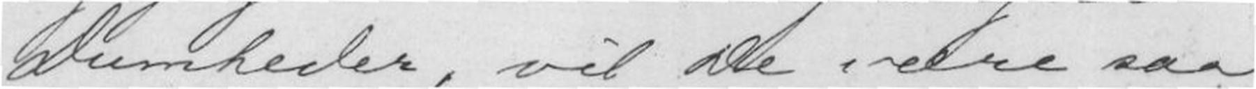Dumheder, vil De være saa
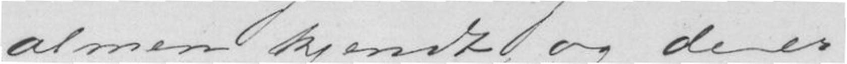almen kjendt og de er
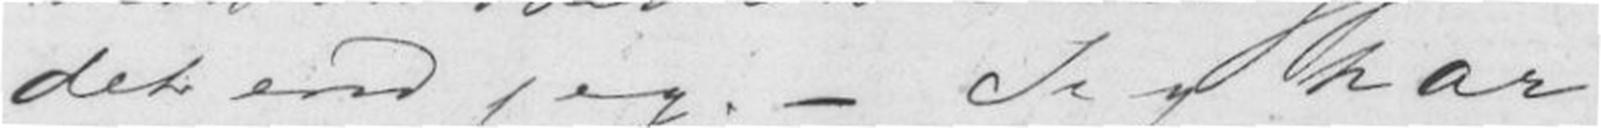den enn jeg.- Jeg har
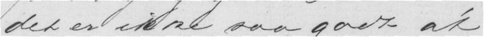det er ikke saa godt at
